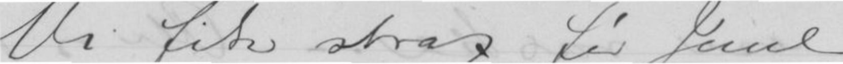Vi fik strax før Juul
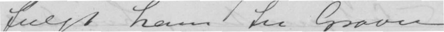fulgt ham til Graven.
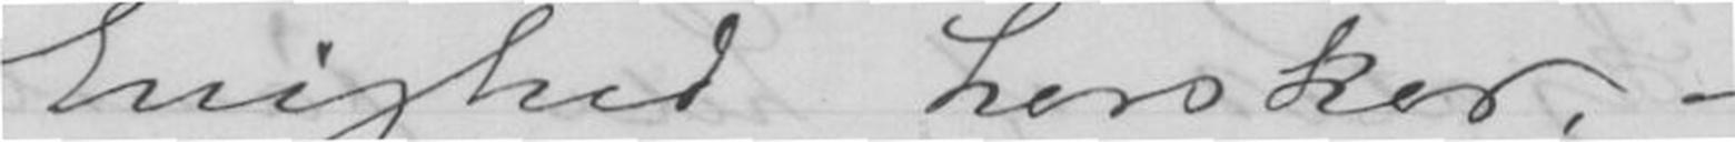Enighed hersker, og
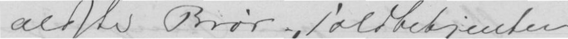ældre Bròr Toldbetjenten
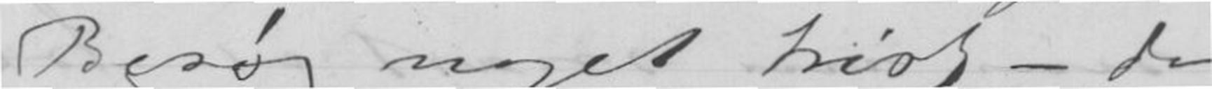Besøg noget trist - dog
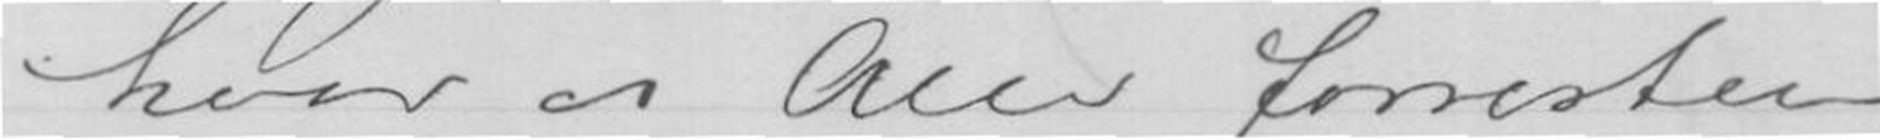hvor at Alle forresten
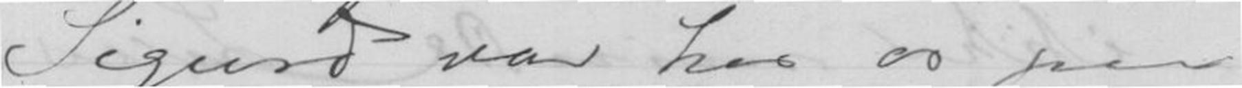Sigurd var hos os paa
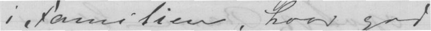i Familien, hvor god
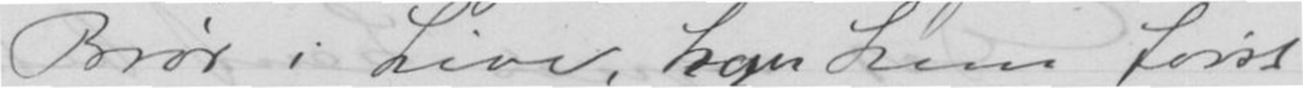Brór i Live, han kom først
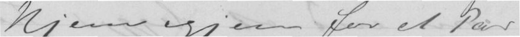Hjem igjen for et par
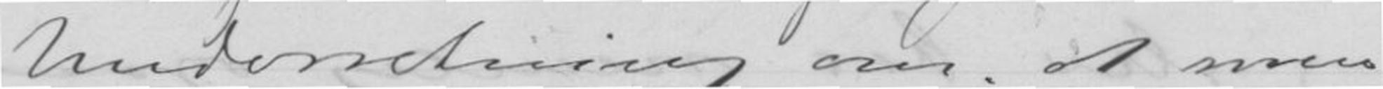Underretning om at min
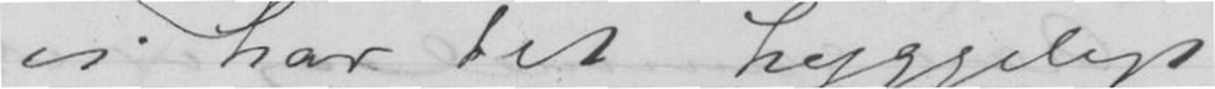vi har det hyggeligt
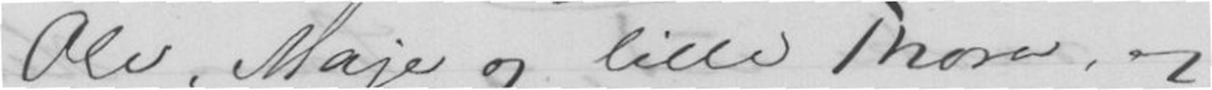Ole, Maje og lille Thora og
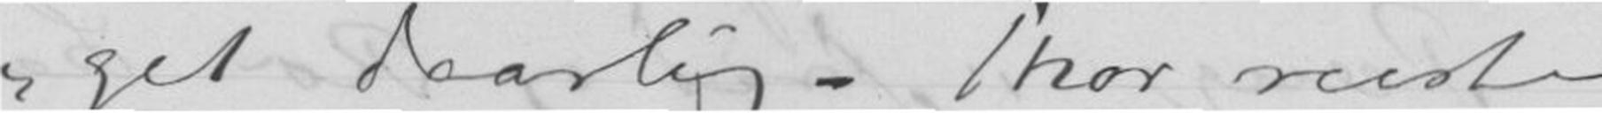get daarlig - Thor reiste
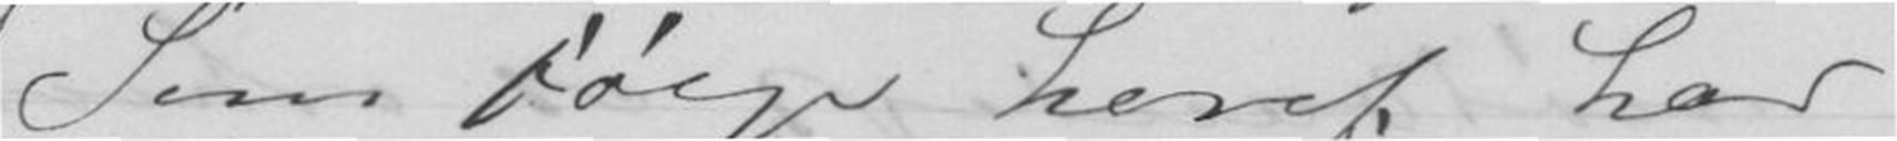Som Følge heraf har
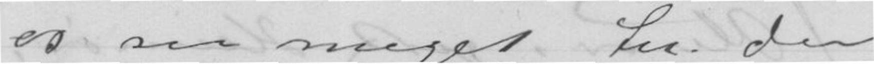os saa meget til der
